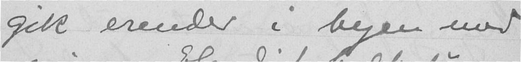gik erender i byen med
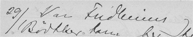29/1 Var Fridheims og
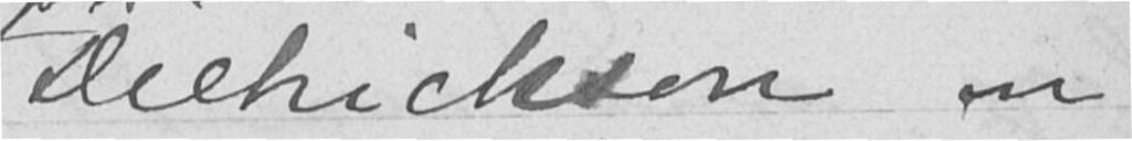Dietrichson om
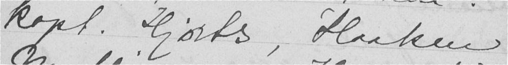kapt. Hjorts, Haaken
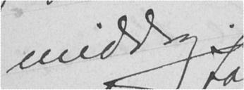middag.
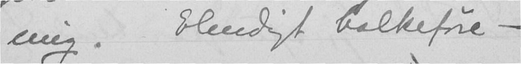mig. Elendigt holkeføre -
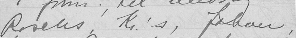Raschs, Kr.'s, Johan,
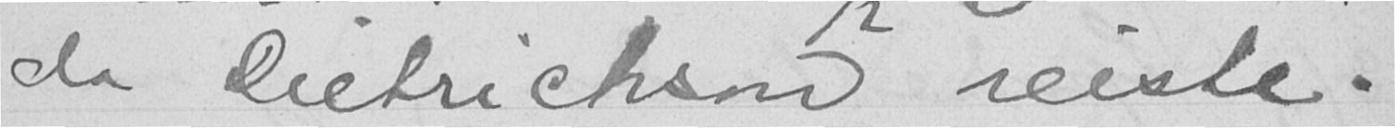da Dietrichson reiste.
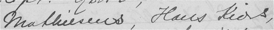Mathiesens, Hans Kiærs,
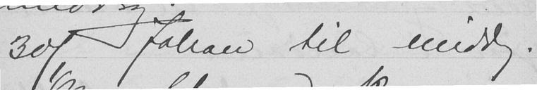30/1 Johan til middag.
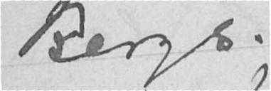Bergs. kapt.
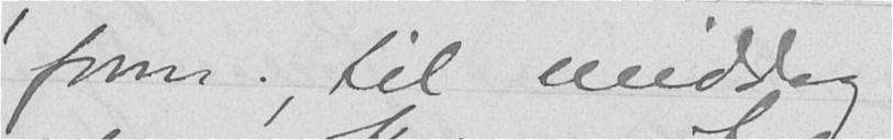form., til middag
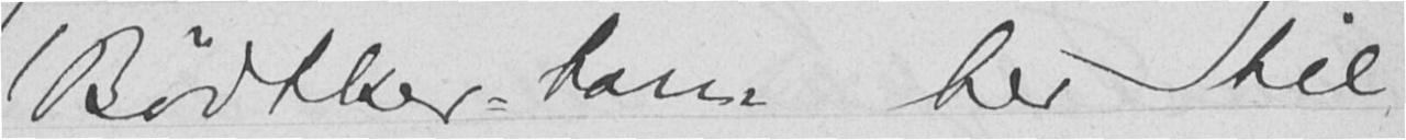Bødtker-barn her til
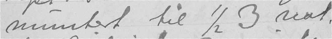muntert til ½  3 nat,
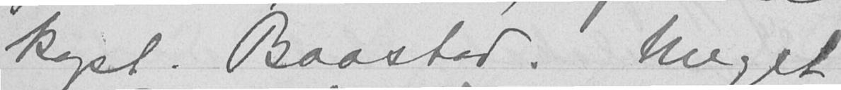kapt. Baastad. Meget
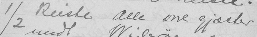1/2 Reiste alle vore gjæster
